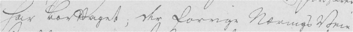har borttaget; der forrige Nærings Veie
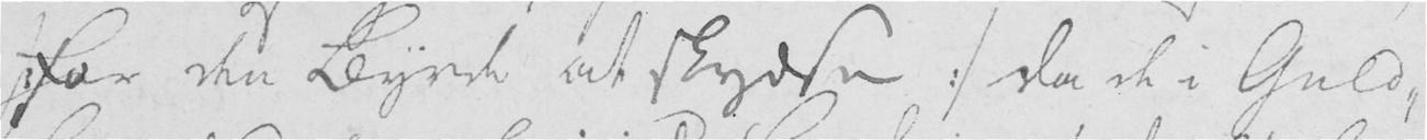for den Byrde at skydse :/ da de i Guld-
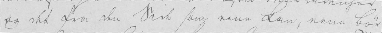og det fra den Side som eene kan, eene bør
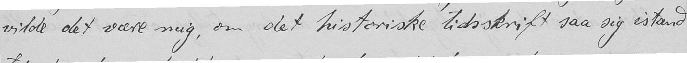vilde det være mig, om det historiske tidsskrift saa sig istand
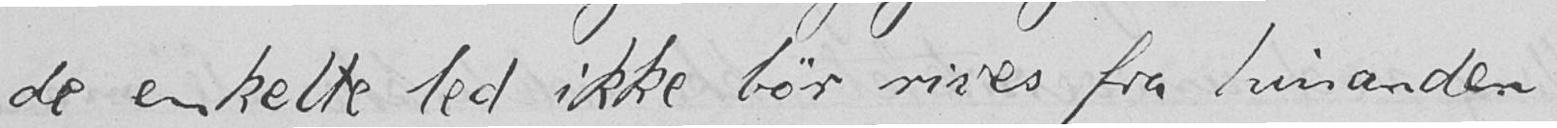de enkelte led ikke bør rives fra hinanden.
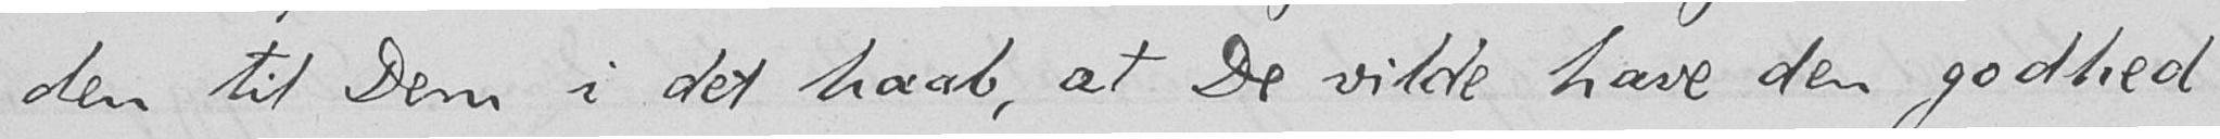den til Dem i det haab, at De vilde have den godhed
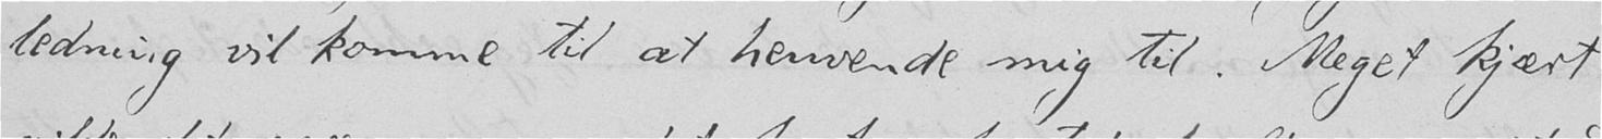ledning vil komme til at henvende mig til. Meget kjært
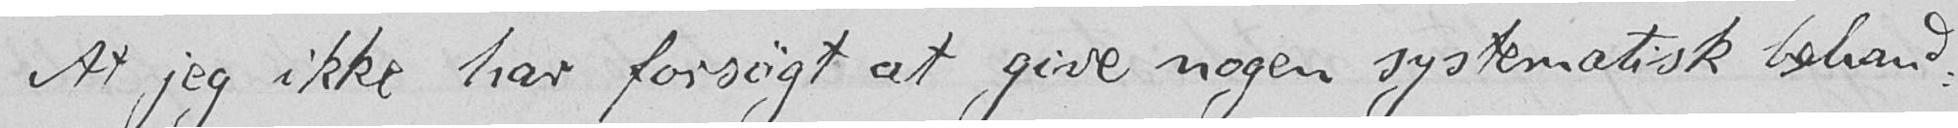At jeg ikke har forsøgt at give nogen systematisk behand-
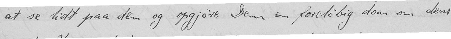at se lidt paa den og opgjøre Dem en foreløbig dom om dens
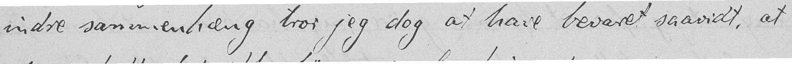indre sammenhæng tror jeg dog at have bevaret saavidt, at
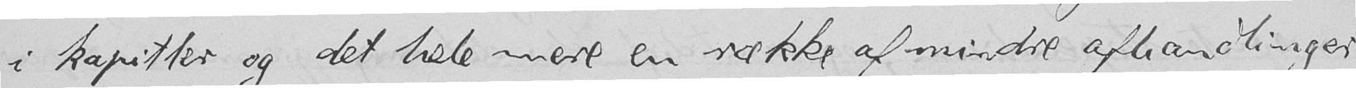i kapitler og det hele mere en række af mindre afhandlinger
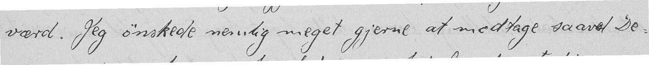værd. Jeg ønskede nemlig meget gjerne at modtage saavel De-
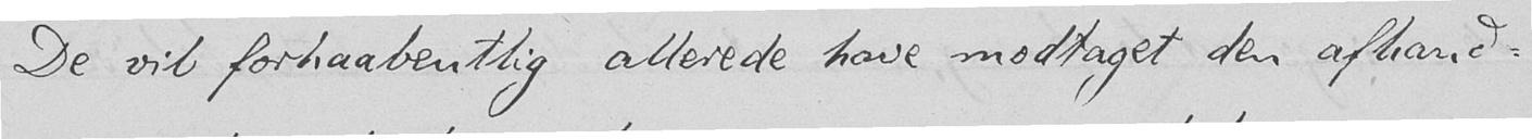De vil forhaabentlig allerede have modtaget den afhand-
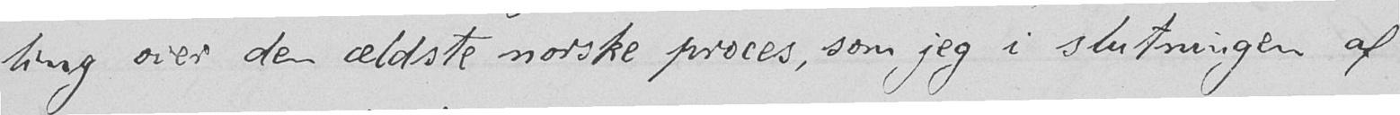ling over den ældste norske proces, som jeg i slutningen af
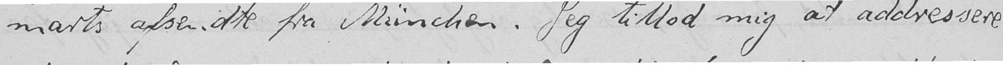marts afsendte fra München. Jeg tillod mig at addressere
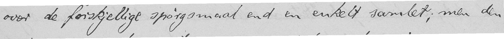over de forskjellige spørgsmaal end en enkelt samlet; men den
